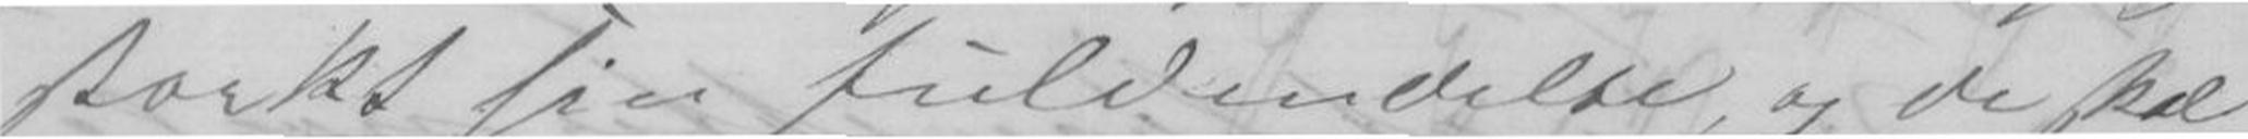stærkt sin fuldendelse, og de skal
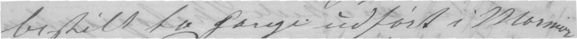bestilt to Gange udført i Marmor
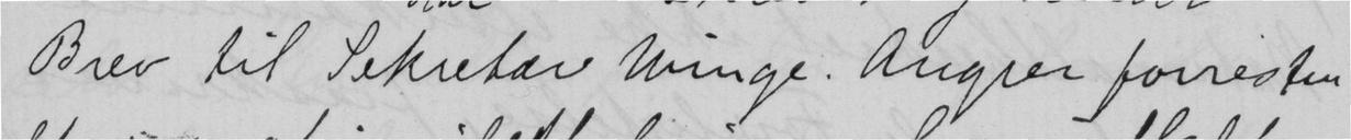Brev til Sekretær Winge. Angrer forresten
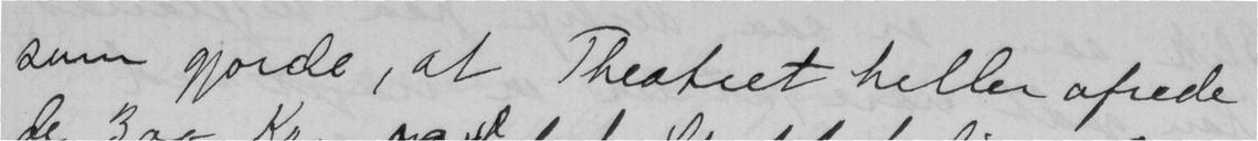som gjorde, at Theatret heller ofrede
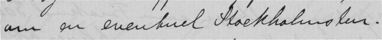om en eventuel Stockholmstur.
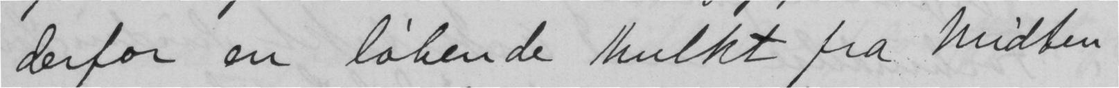derfor en løbende Mulkt fra midten
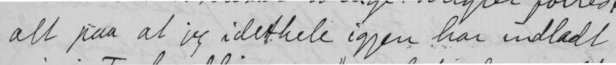alt paa at jeg idethele igjen har indladt
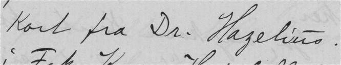Kort fra Dr. Hagelius.
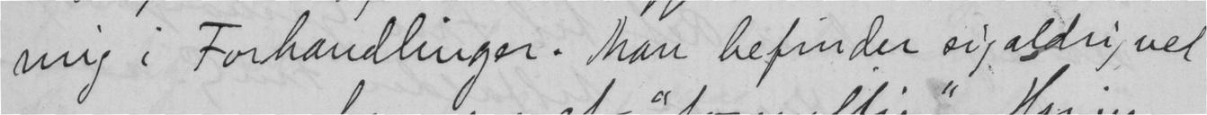mig i Forhandlinger. Man befinder sig aldrig vel
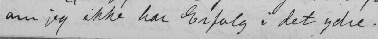om jeg ikke har Erfolg i det ydre.
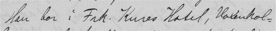Han bor i Frk. Kures Hotel, Voxenkol-
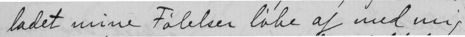ladet mine Følelser løbe af med mig
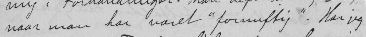naar man har været "fornuftig". Har jeg
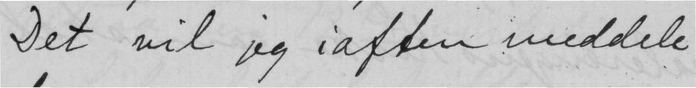Det vil jeg iaften meddele
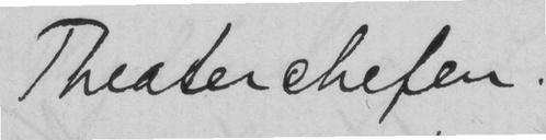Theaterchefen.
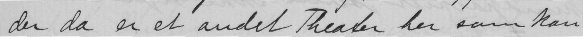der da er et andet Theater her som kan
